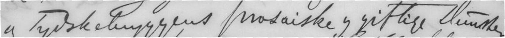og Tydskebryggens prosaiske og giftige Dunster,
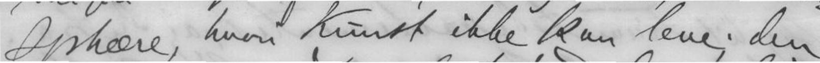sphære, hvor Kunst ikke kan leve; den
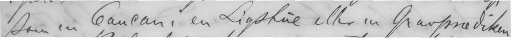som en Cancan i en Ligstue eller en Gravprædiken
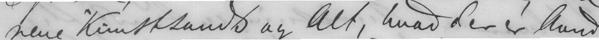rene "Kunstsands og Alt, hvad der er Aand,
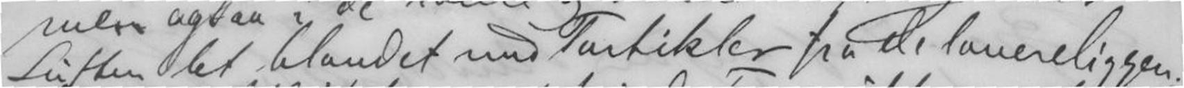Luften let blandet med Partikler fra de lavereliggen-
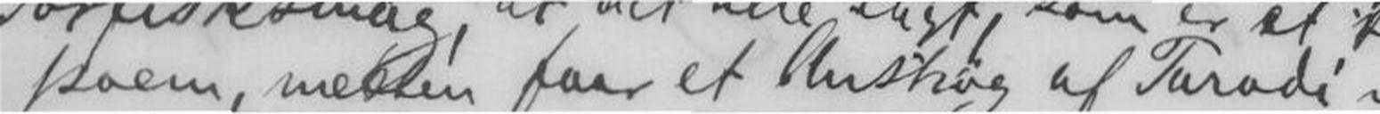poem, næsten faar et Anstrøg af Parodi mod Digteren Villie
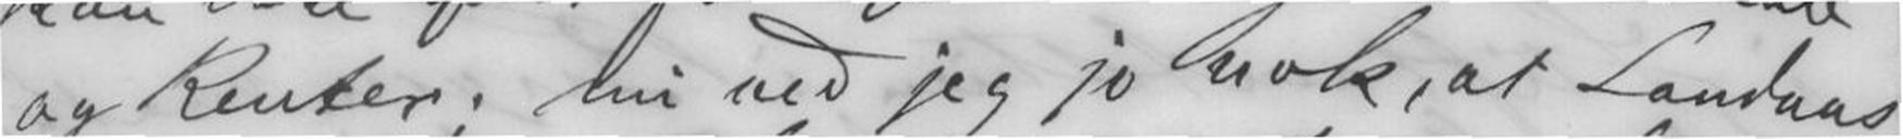og Renter; nu ved jeg jo nok, at Landaas
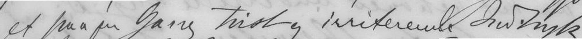et paa en Gang trist og irriterende Indtryk
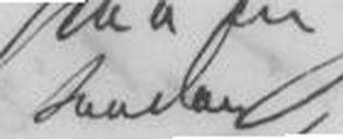saadan
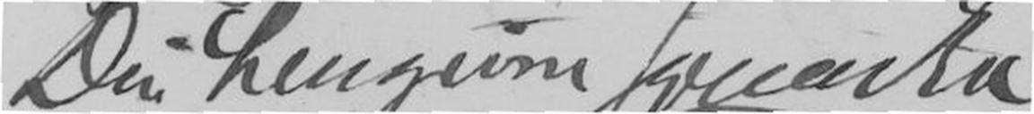Din hengivne Jonas Lie.
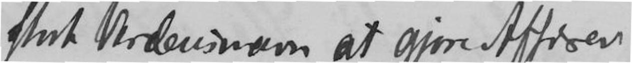stort Verdensnavn at gjøre Affærer
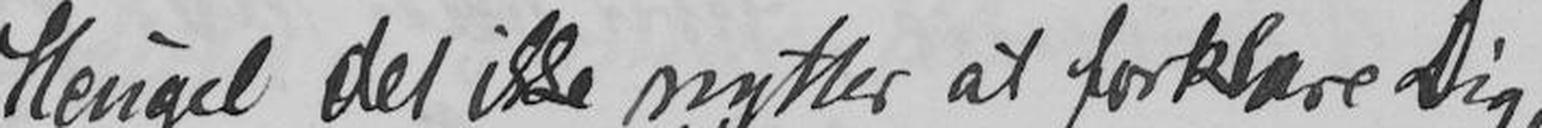Hengel det ikke nytter at forklare Dig,
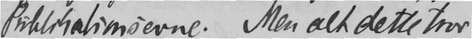Publikationsevne. Men alt dette tror
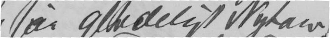Og saa glædeligt Nytaar!
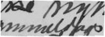gammeldagse
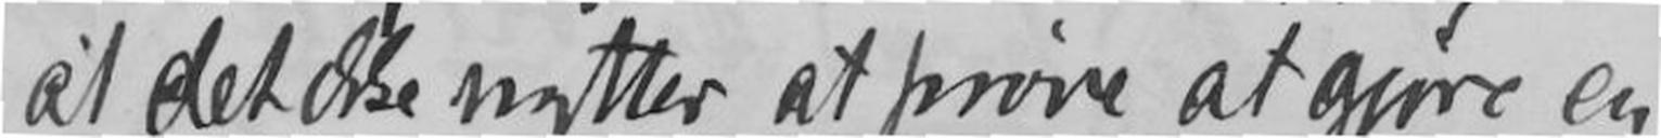at det ikke nytter at prøve at gjøre en
